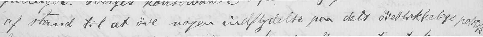af stand til at øve nogen indflydelse paa dets øieblikkelige politik
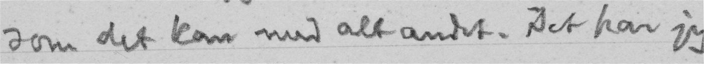som det kan med alt andet. Det har jeg
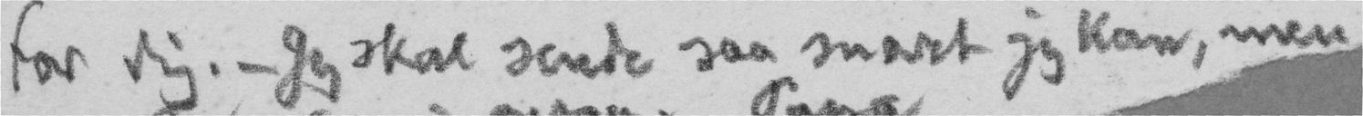for dig. - Jeg skal sende saa snart jeg kan, men
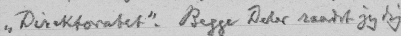"Direktoratet". Begge Deler raadet jeg dig
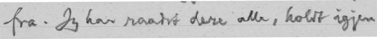fra. Jeg har raadet dere alle, holdt igjen
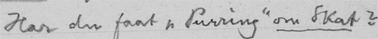Har du faat "Purring" om Skat?
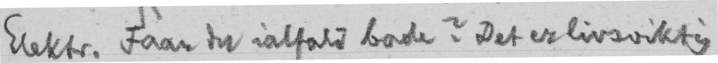Elektr. Faar du ialfald bade. Det er livsviktig
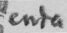enda
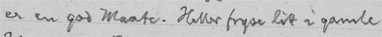er en god Maate. Heller fryse litt i gamle
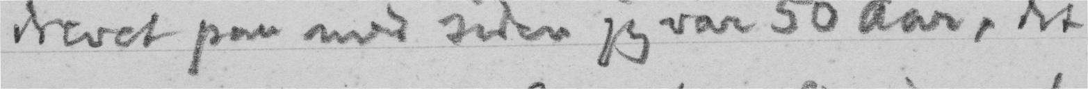drevet paa med siden jeg var 50 Aar, det
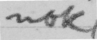nok
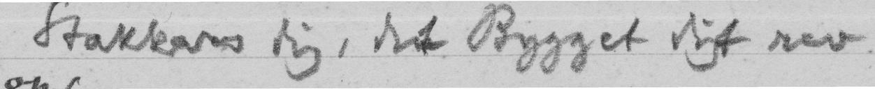Stakkars dig, det Bygget ditt rev
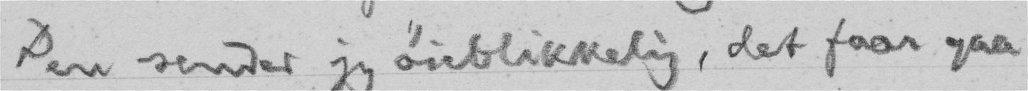Den sender jeg øieblikkelig, det faar gaa
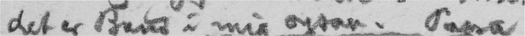det er Bund i mig ogsaa. Papa
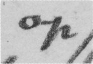op
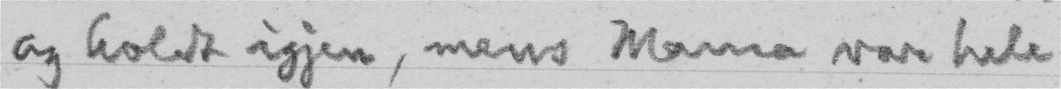og holdt igjen, mens Mama var hele
